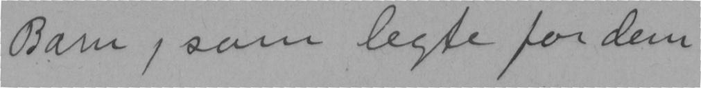Barn, som legte for dem
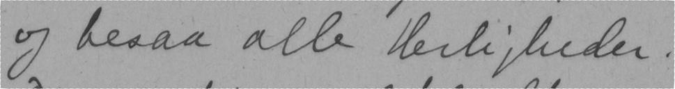og besaa alle Herligheder.
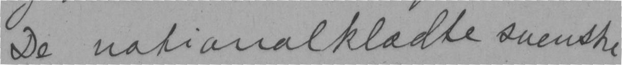De nationalklædte svenske
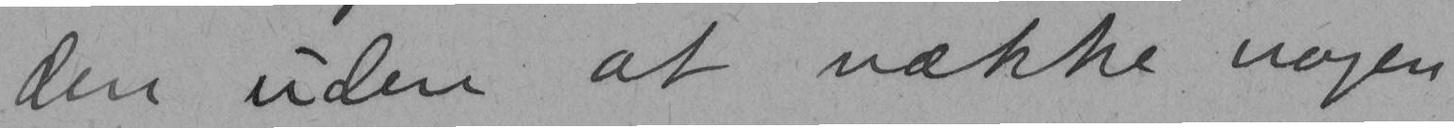den uden at vække nogen
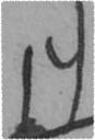14
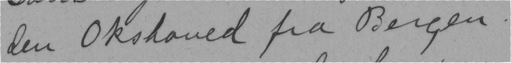den Okshoved fra Bergen.
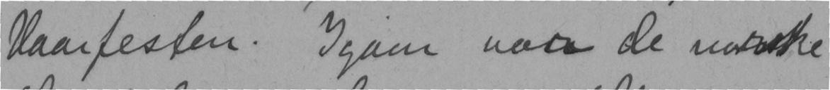Vaarfesten. Igaar var de norske
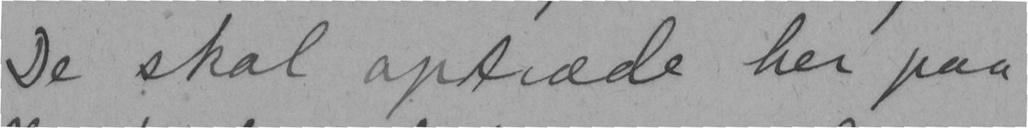De skal optræde her paa
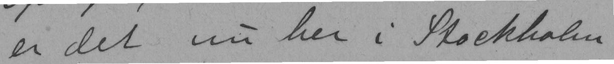er det nu her i Stockholm
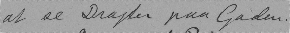at se Dragter paa Gaden.
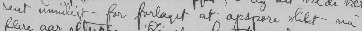rent umuligt for forlaget at opspore slikt nu
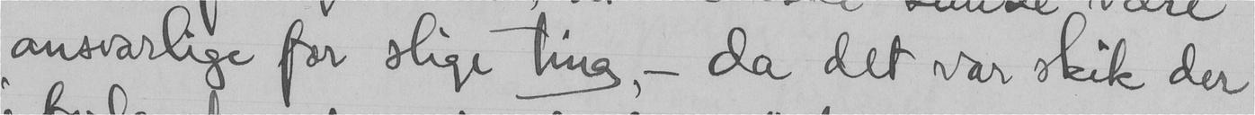ansvarlige for slige ting, - da det var skik der
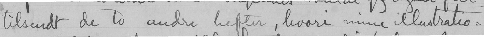tilsendt de to andre hefter, hvori mine illestratio-
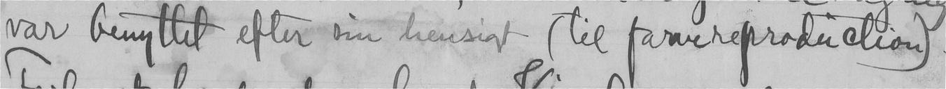var benyttet efter sin hensigt (til farvereproduction).
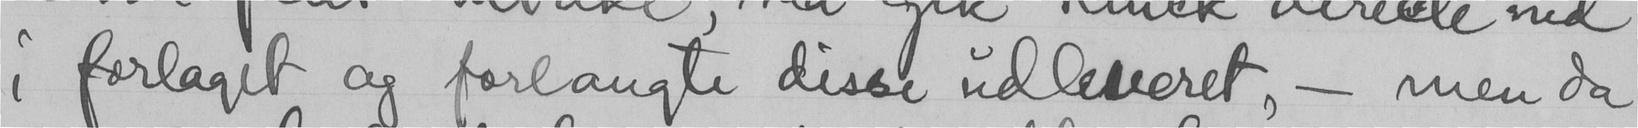i forlaget og forlangte disse udleveret, - men da
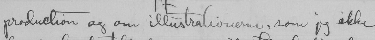production og om illustrationerne, som jeg ikke
